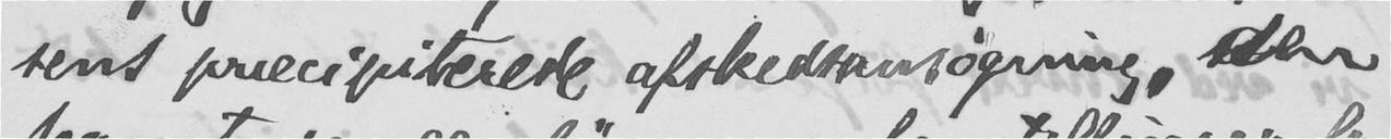sens præcipiterede afskedsansøgning, den
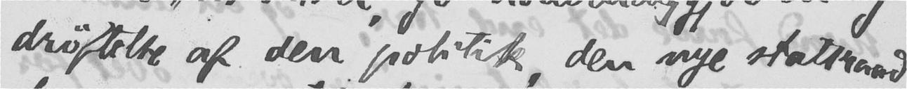drøftelse af den politik, den nye statsraad
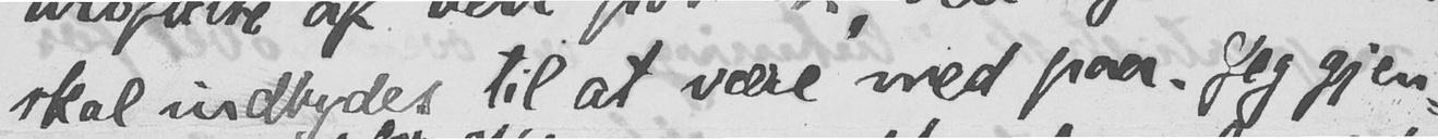skal indbydes til at være med paa. Jeg gjen-
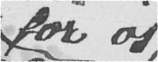for os
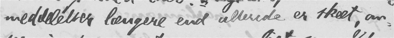meddelelser længere end allerede er skeet, an-
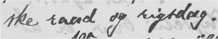ske raad og rigsdag.
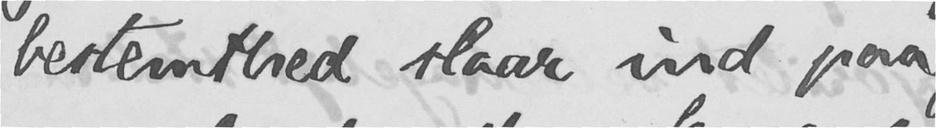bestemthed slaar ind paa
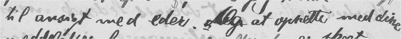til ansigt med eder. Og at opsætte med dine
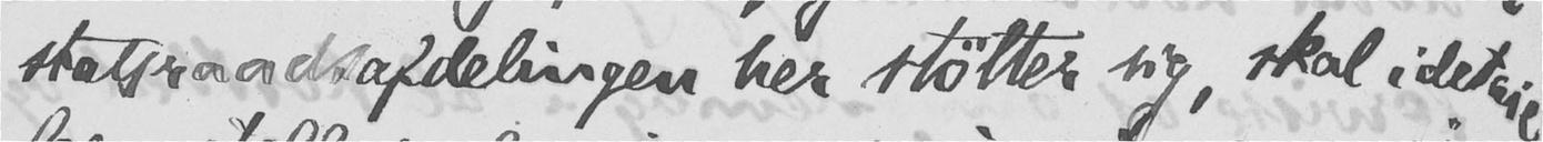statsraadsafdelingen her støtter sig, skal i detail
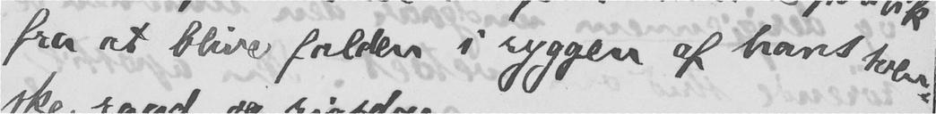fra at blive falden i ryggen af hans sven-
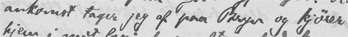ankomst tager jeg af paa Bryn og kjører
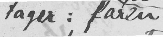tager: faren
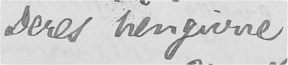Deres hengivne
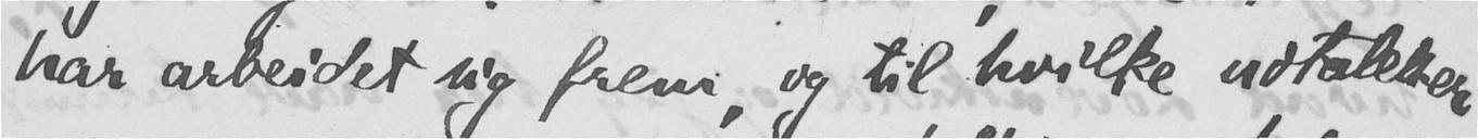har arbeidet sig frem, og til hvilke udtalelser
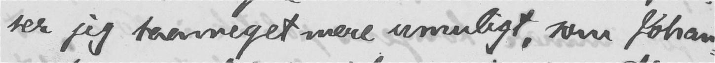ser jeg saameget mere umuligt, som Johan-
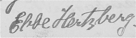Ebbe Hertzberg.
